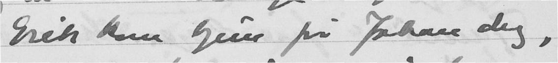Erik kom hjem før Johan drog,
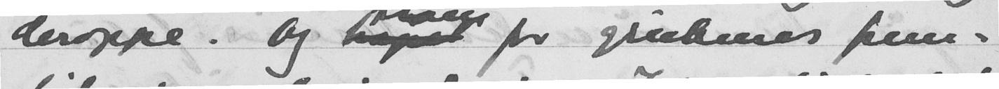deroppe. Og  lenge for grubenes frem-
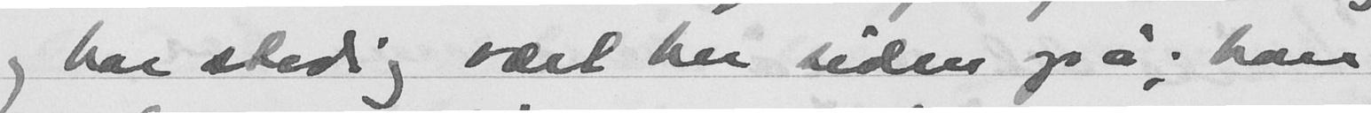og har stedig vært her siden også; han
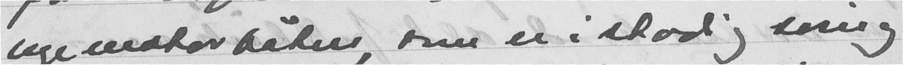lege motor båten, som er i stadig sving
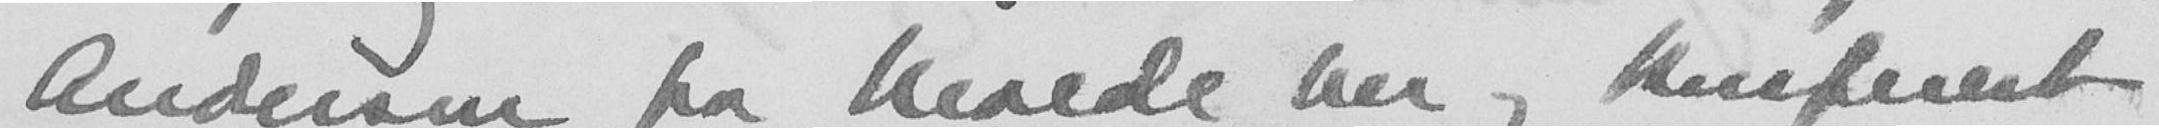Andersen fra Molde her og kusferest
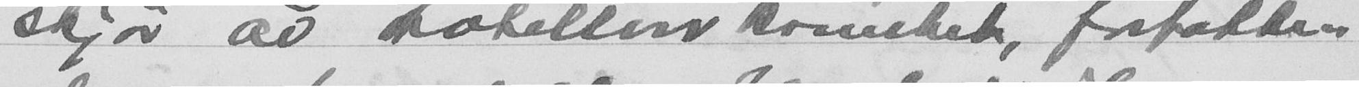skjør av hotellokomihiet, forfatter-
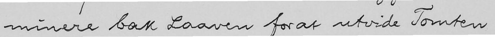minere bak Laaven forat utvide Tomten
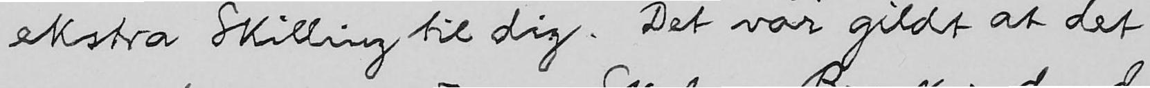ekstra Skilling til dig. Det var gildt at det
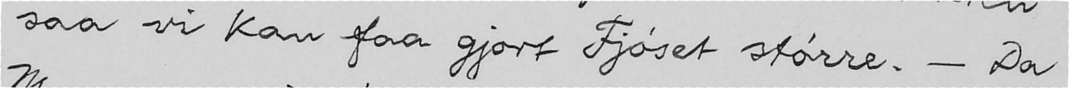saa vi kan faa gjort Fjøset større. - Da
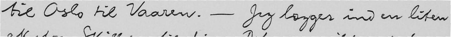til Oslo til Vaaren. - Jeg lægger ind en liten
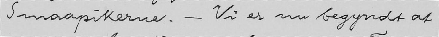Smaapikerne. - Vi er nu begyndt at
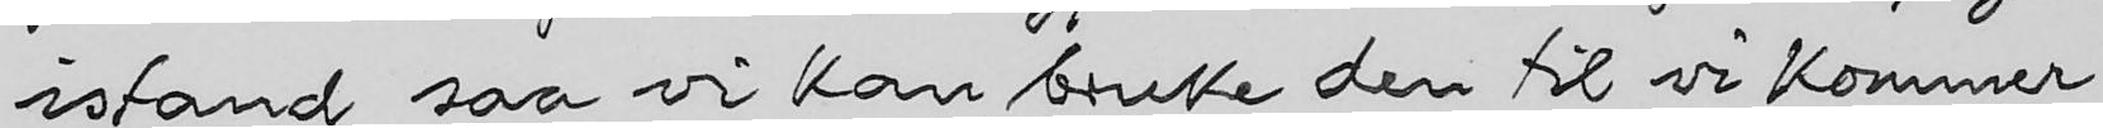istand saa vi kan bruke den til vi kommer
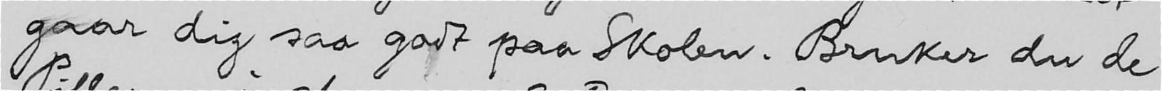gaar dig saa godt paa Skolen. Bruker du de
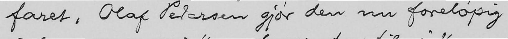faret, Olaf Petersen gjør den nu foreløpig
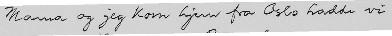Mama og jeg kom hjem fra Oslo hadde vi
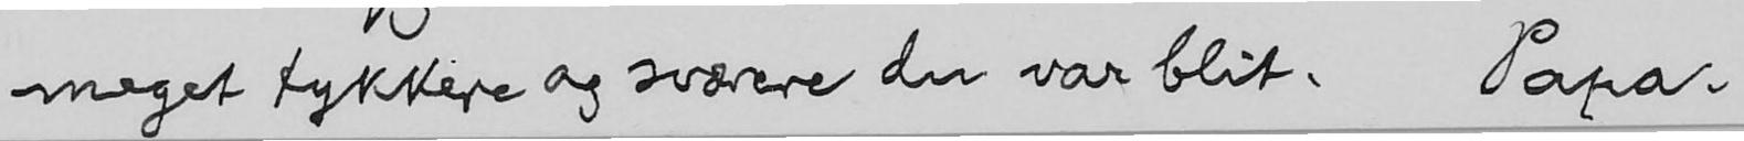meget tykkere og sværere du var blit. Papa.
